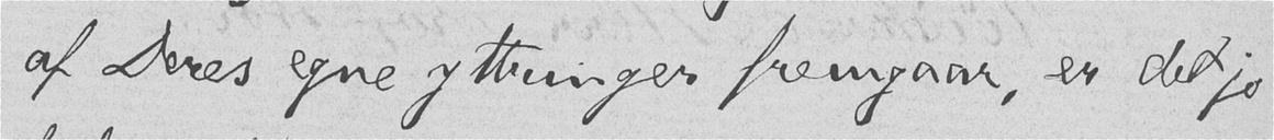af Deres egne yttringer fremgaar, er det jo
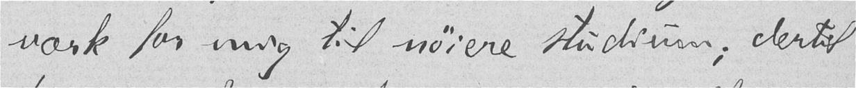værk for mig til nøiere studium; dertil
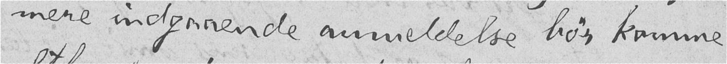mere indgaaende anmeldelse bør komme
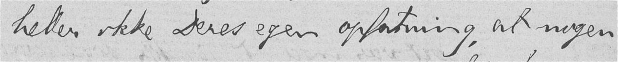heller ikke Deres egen opfatning, at nogen
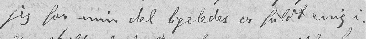jeg for min del ligeledes er fuldt enig i.
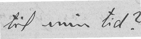til min tid?
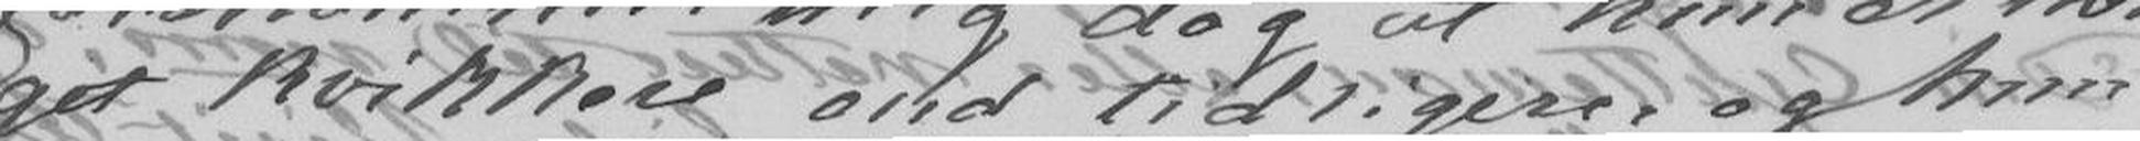get kvikkere end tidligere, og hun
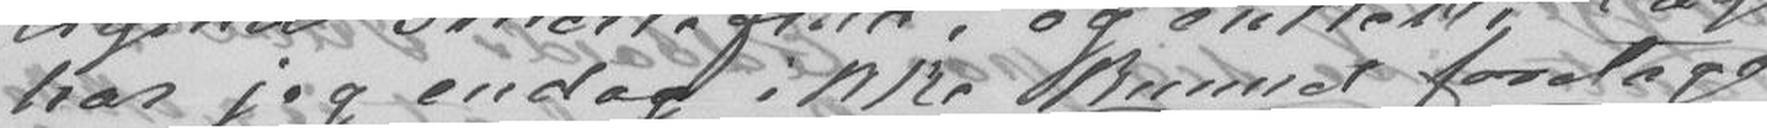har jeg endog ikke kunnet foretage
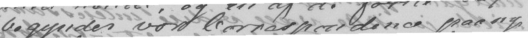begynder vor Correspondence paany.
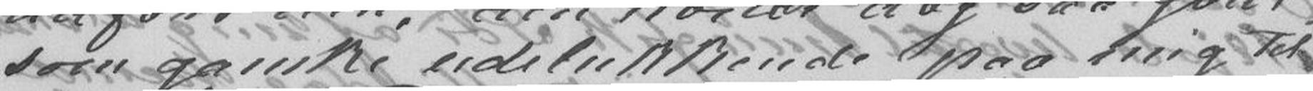som ganske udelukkende paa mig til
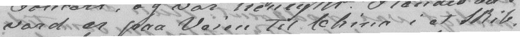vard er paa Veien til China i et Skib
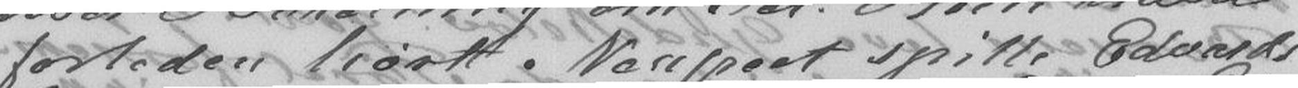forleden hørt Neupert spille Edvards
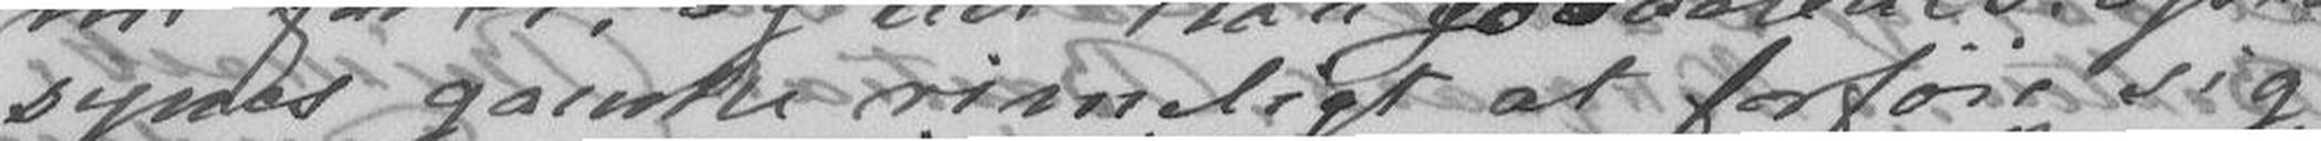synes ganske rimeligt at forføie sig
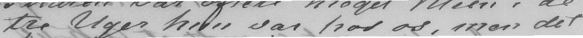tre Uger hun var hos os, men det
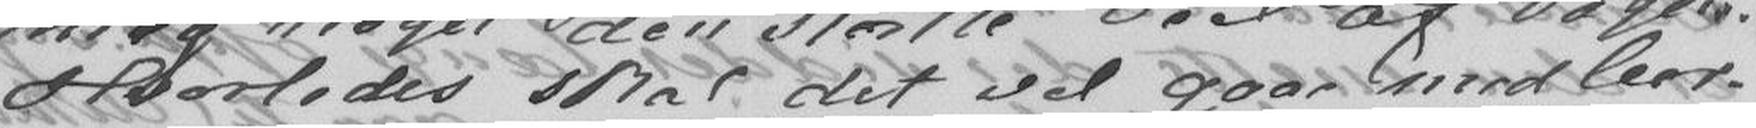Hvorledes skal det vel gaae med Cor-
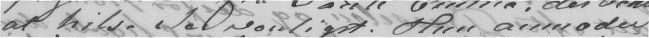at hilse Jer venligst. Hun anmoder
